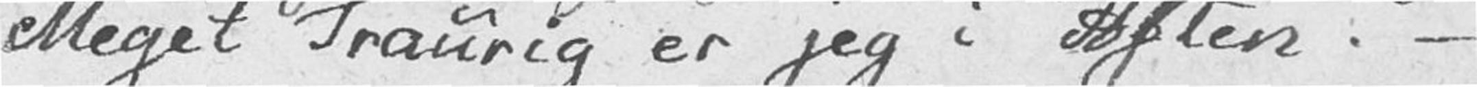Meget Traurig er jeg i Aften. –
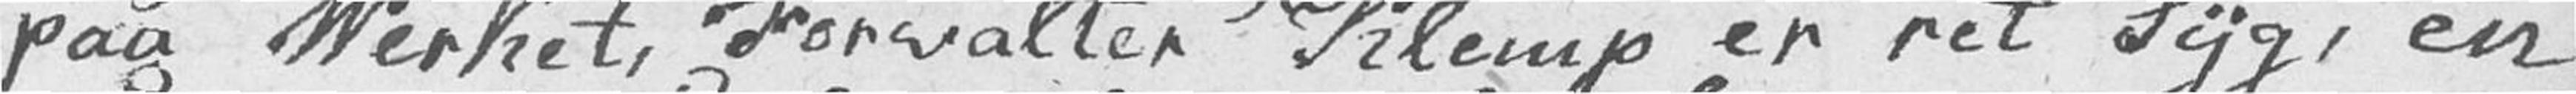paa Verket, Forvalter Klemp er ret Syg, en
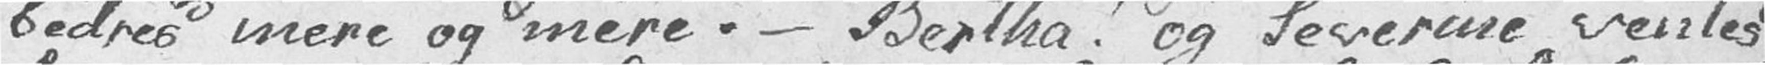bedres mere og mere. – Bertha! og Severine ventes
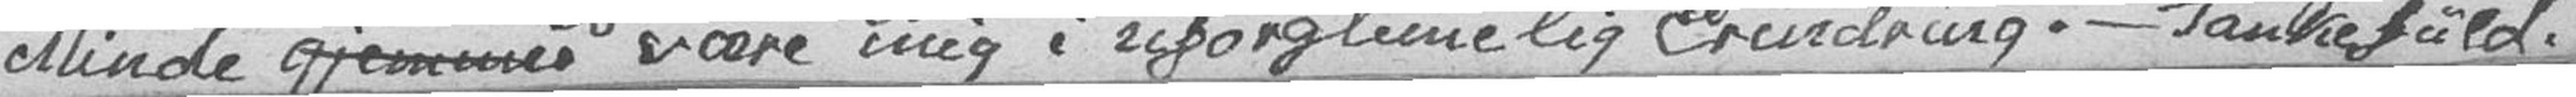Minde gjemmes være mig i uforglemelig Erindring. – Tankefuld.
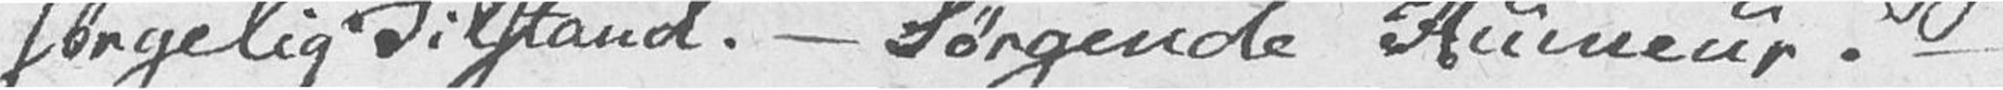sørgelig Tilstand. – Sørgende Humeur. –
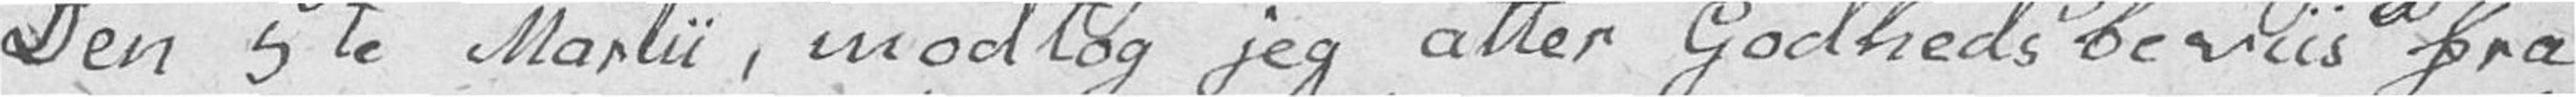Den 5te Martii, modtog jeg atter Godheds beviis fra
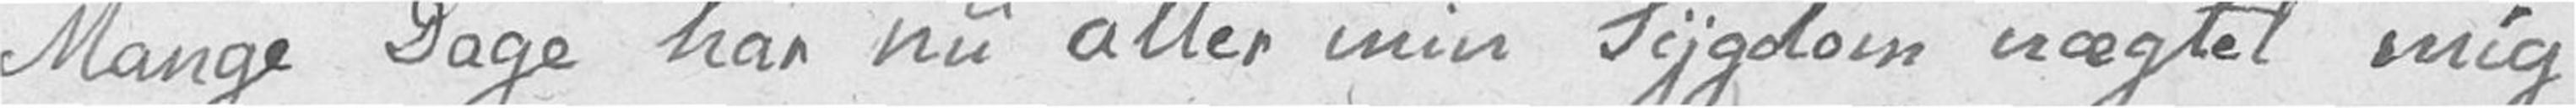Mange Dage har nu atter min Sygdom nægtet mig
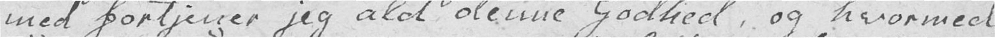med fortjener jeg ald denne Godhed, og hvormed
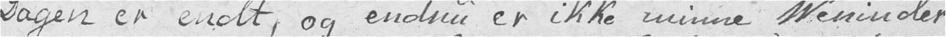Dagen er endt, og endnu er ikke minne Veninder
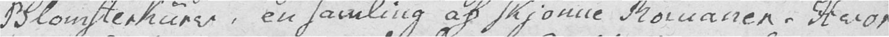Blomsterkurv, en samling af skjønne Romaner. Hvor
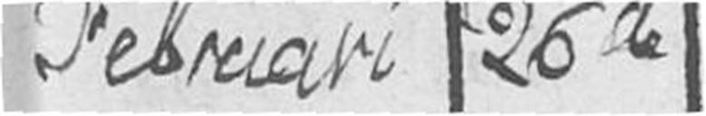Februari 26de
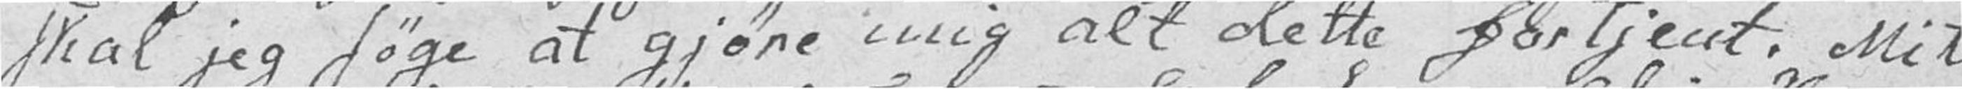skal jeg søge at gjøre mig alt dette fortjent. Mit
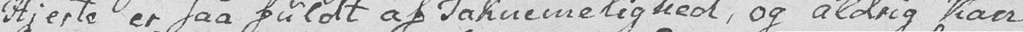Hjerte er saa fuldt af Taknemmelighed, og aldrig kan
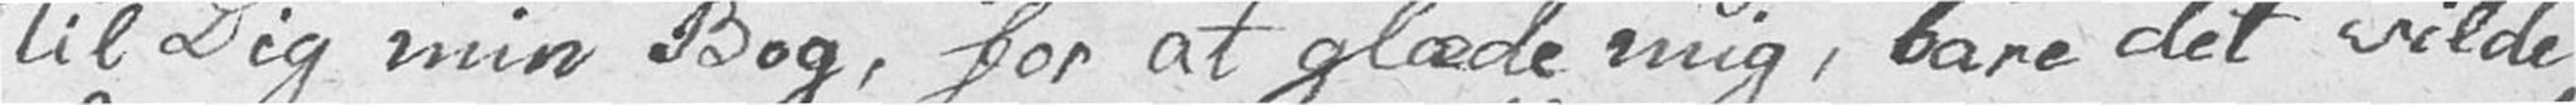til Dig min Bog, for at glæde mig, bare det vilde
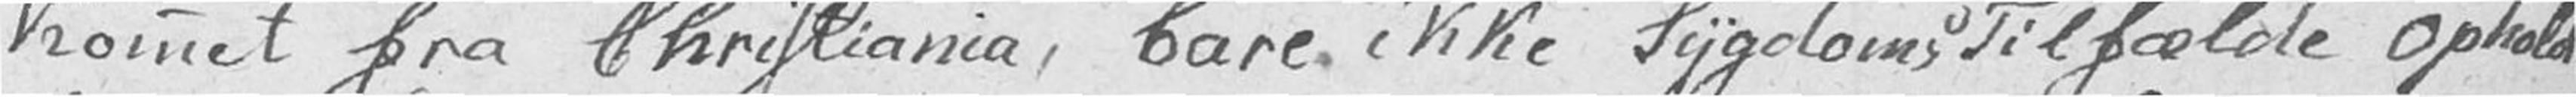kommet fra Christiania, bare ikke Sygdoms Tilfælde opholdt
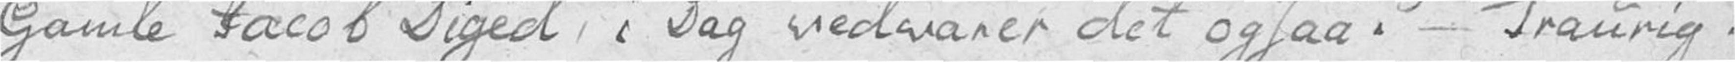Gamle Jacob Diged, i Dag vedvarer det ogsaa. – Traurig.
